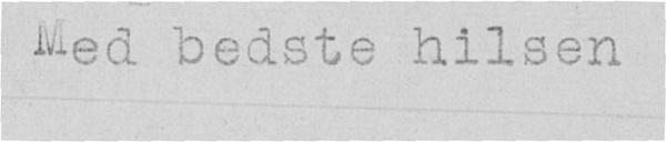Med bedste hilsen
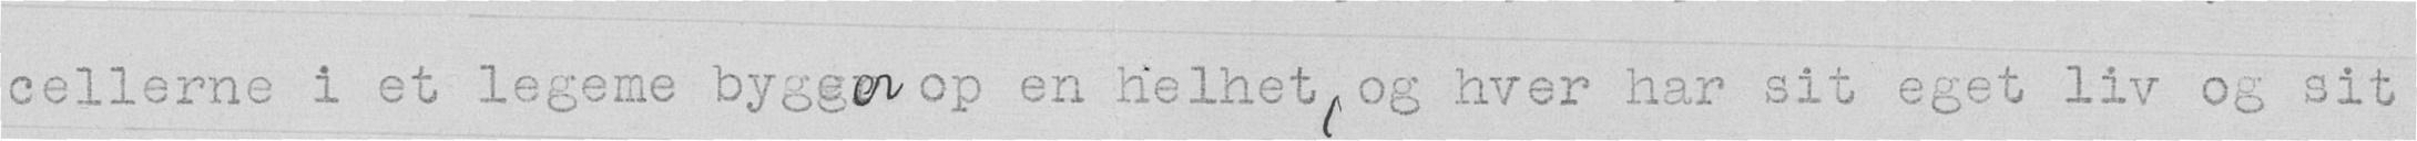cellerne i et legeme bygger op en helhet, og hver har sit eget liv og sit
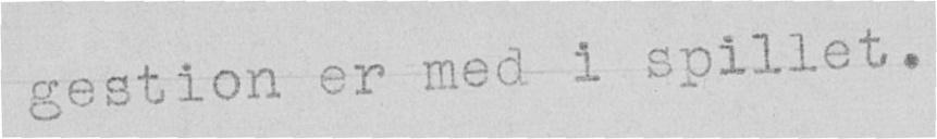gestion er med i spillet.
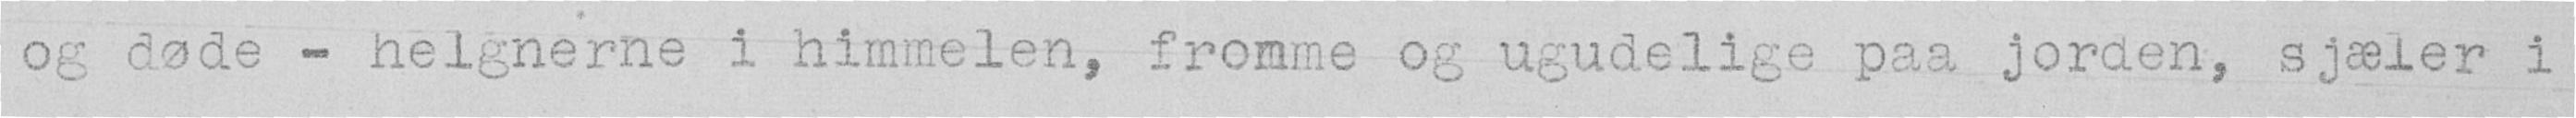og dede - helgnerne i himmelen, fromme og ugudelige paa jorden, sjæler i
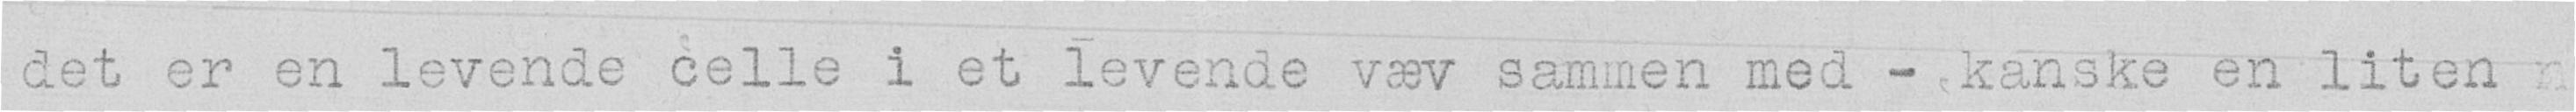det er en levende celle i et levende væv sammen med - kanske en litenn
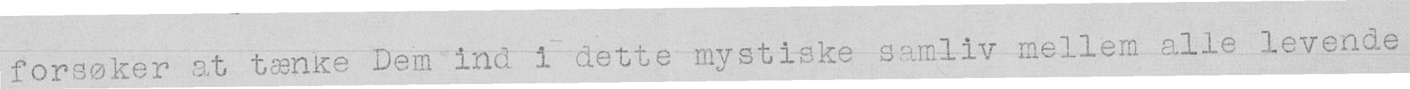forsoker at tænke Dem ind i dette mystiske samliv mellem alle levende
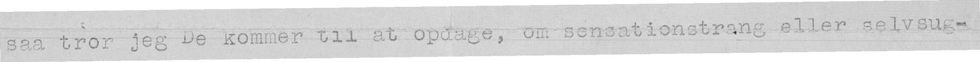saa tror jeg De kommer til at opdage, om sensationstrang eller selvsug¬
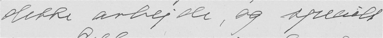dette arbejde, og specielt
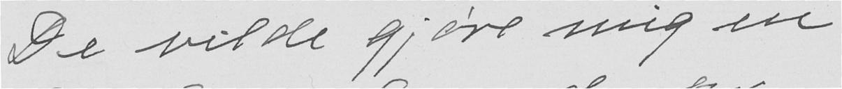De vilde gjøre mig en
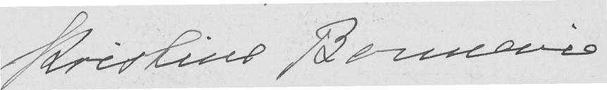Kristine Bonnevie
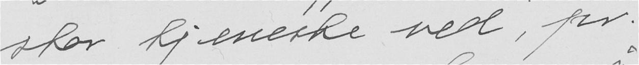stor tjeneste ved, pr.
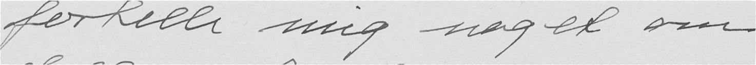fortelle mig noget om
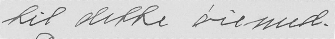til dette øiemed.
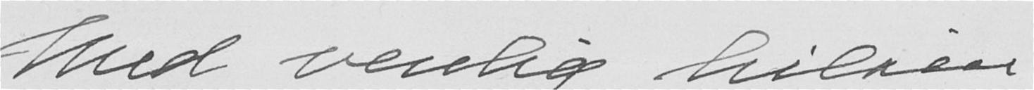Med venlig hilsen
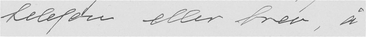telefon eller brev, å
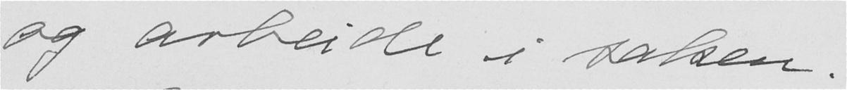og arbeide i saken.
